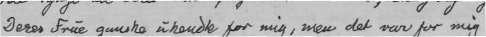Deres Frue ganske ukendte for mig, men det var for mig
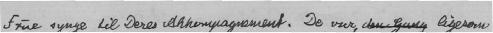Frue synge til Deres Akkompagnement. De var,den Gang ligesom
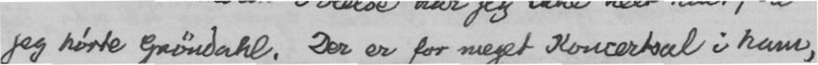jeg hørte Grøndahl. Der er for meget Koncertsal i ham,
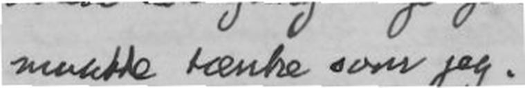maatte tænke som jeg.
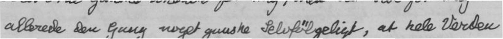allerede den Gang noget ganske Selvfølgeligt, at hele Verden
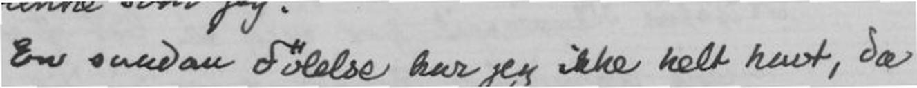En saadan Følelse har jeg ikke helt havt, da
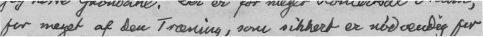for meget af den Træning, som sikkert er nødvændig for
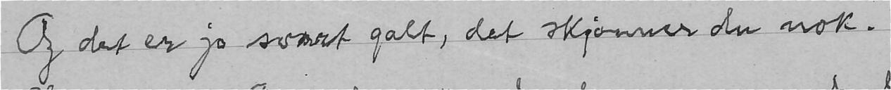Og det er jo svært galt, det skjønner du nok.
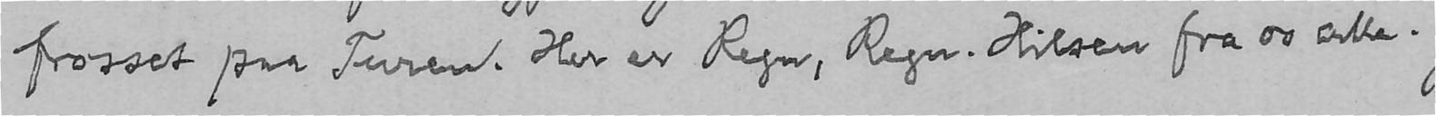frosset paa Turen. Her er Regn, Regn. Hilsen fra os alle.
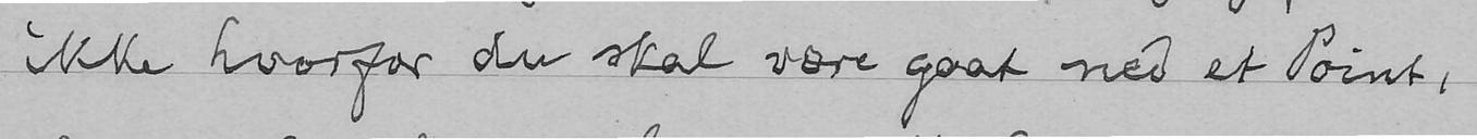ikke hvorfor du skal være gaat ned et Point,
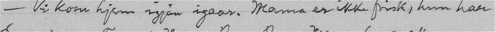- Vi kom hjem igjen igaar. Mama er ikke frisk, hun har
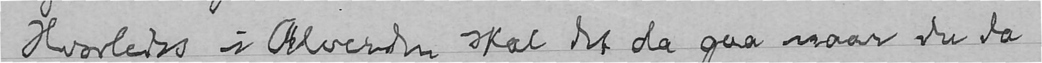Hvorledes i Alverden skal det da gaa naar du da
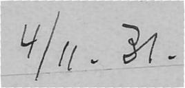4/11. 31.
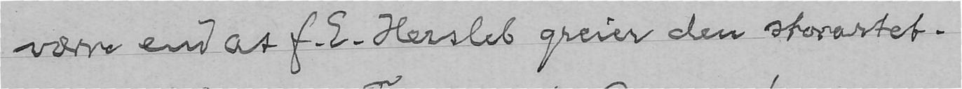værre end at f.E. Hersleb greier den storartet.
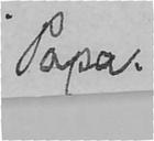Papa.
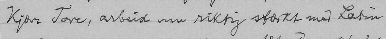Kjære Tore, arbeid nu riktig stærkt med Latin
